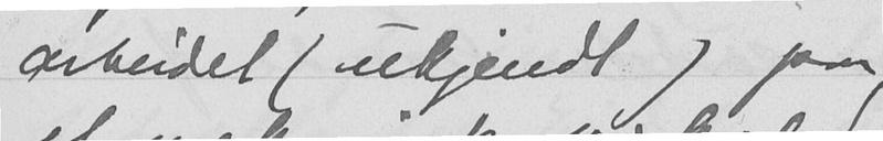arbeidet (ukjendt) paa
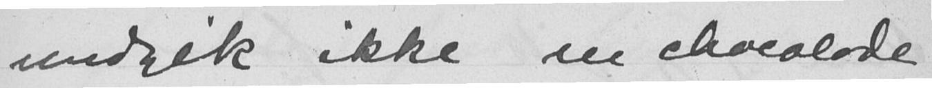undgik ikke en chocolade
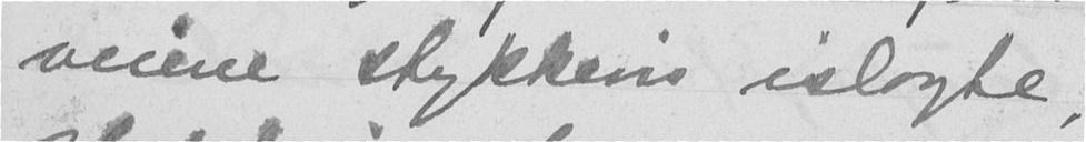veiene stykkevis islagte,
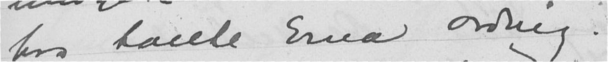hos tante Erna Ording.
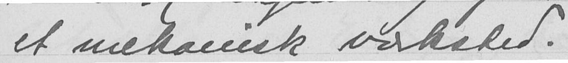et mekanisk værksted.
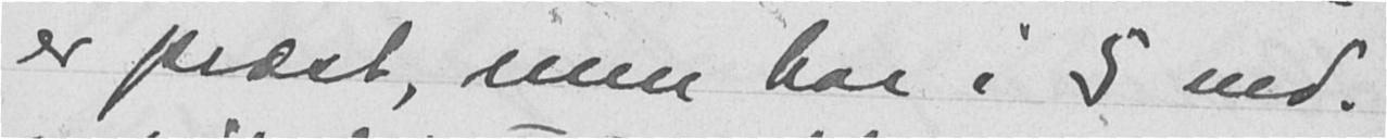er præst, men har i 5 md.
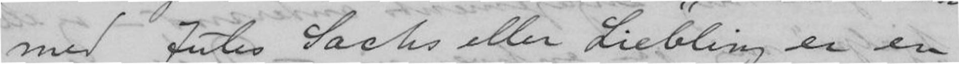med Jules Sachs eller Liebling er en
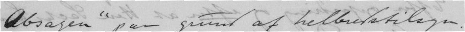"Absagen" paa grund af helbredstilsyn.
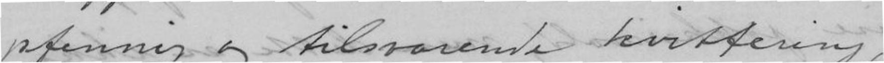phennig og tilsvarende kvittering,
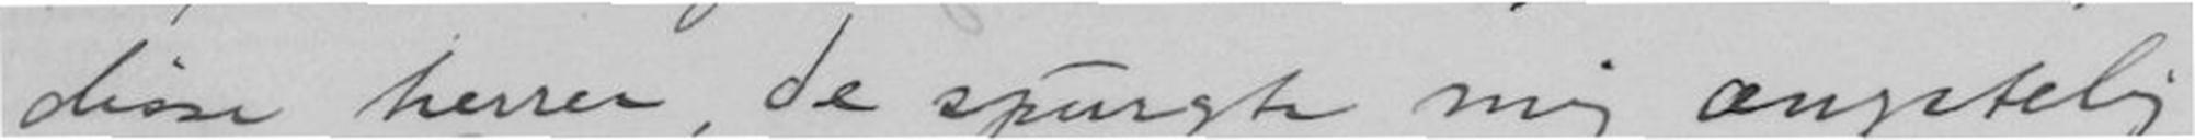disse herrèr, de spurgte mig ængstelig
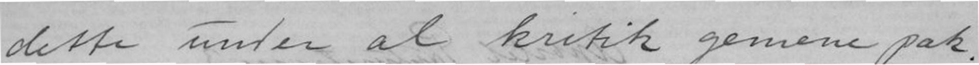dette under al kritik gemene pak.
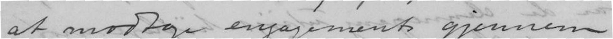at modtage engagements gjennem
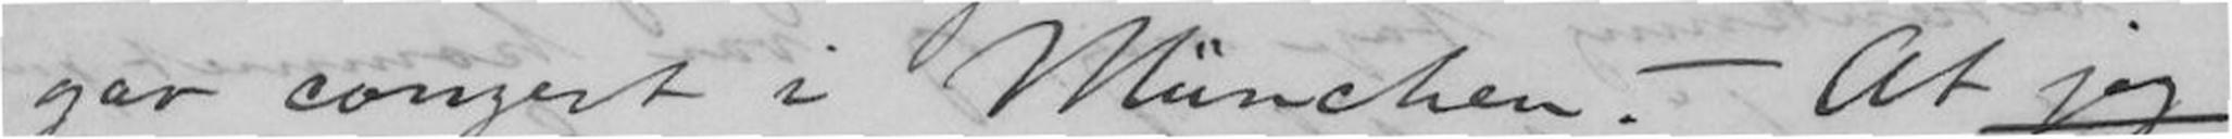gav conzert i München. - At jeg
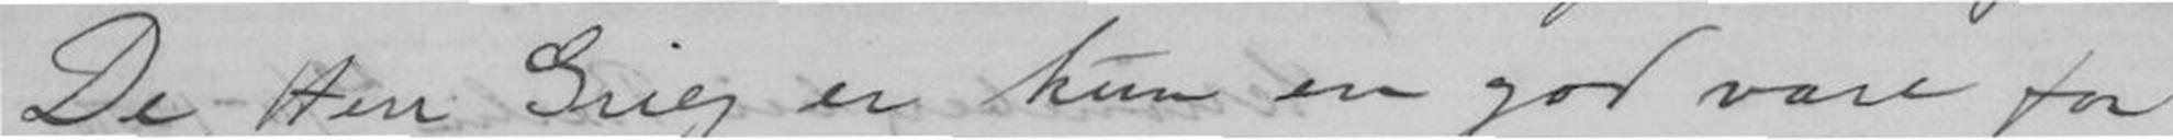De, Herr Grieg er kun en god vare for
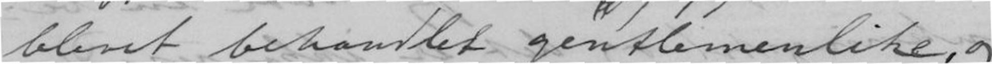blevet behandlet gentlemenlike, og
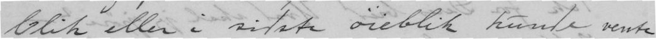blik eller i sidste øieblik kunde vente
